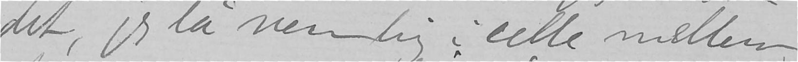det, jeg lå nemlig i celle mellem
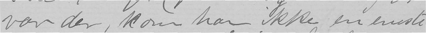var der, kom han ikke en eneste
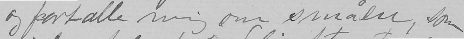og fortælle mig om småen, som
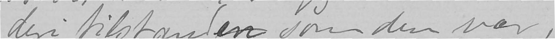deri tilstanden som den var,
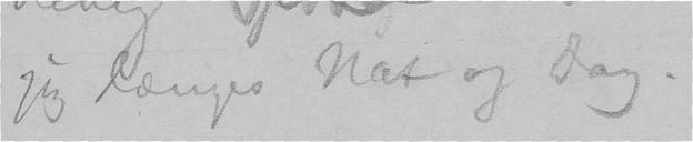jeg længes Nat og Dag.
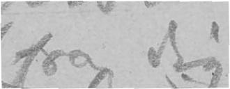fra dig
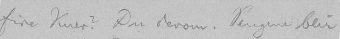fire Kuer? Du derom. Pengene blir
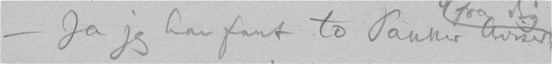- Ja jeg har faat to Pakker Aviser
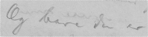Og bare du er
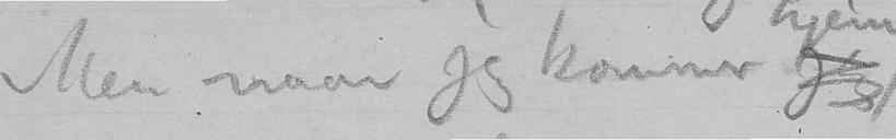Men naar jeg kommer je
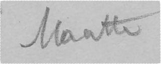Maatte
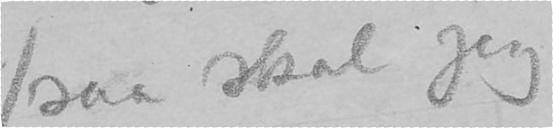saa skal jeg
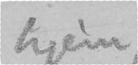hjem
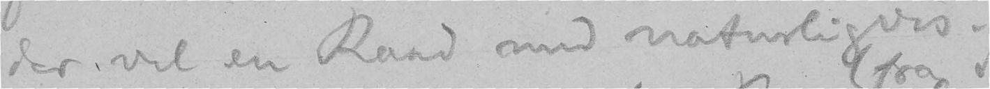der vil en Raad med naturligvis.
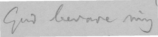Gud bevare mig
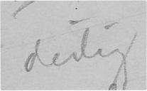deilig
